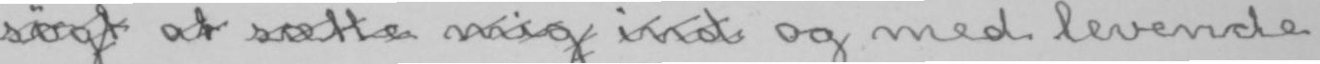søgt at sætte mig ind og med levende
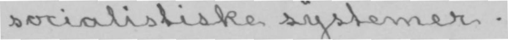socialistiske systemer.
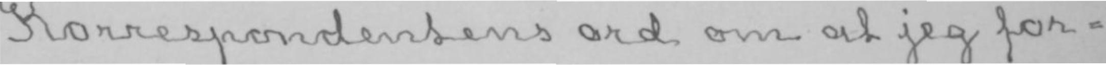Korrespondentens ord om at jeg for-
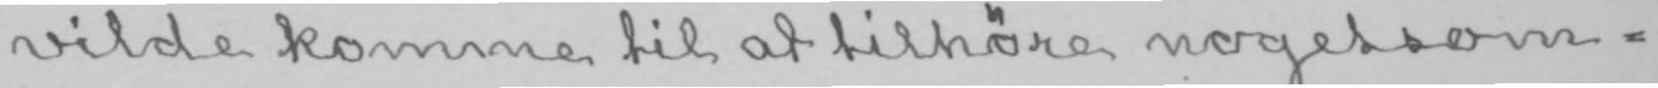vilde komme til at tilhøre nogetsom-
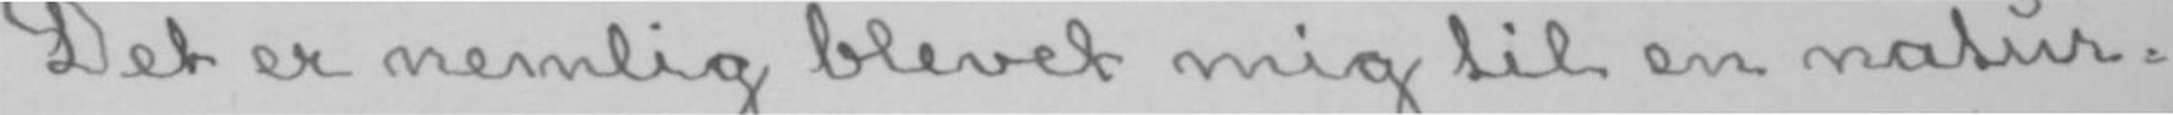Det er nemlig blevet mig til en natur-
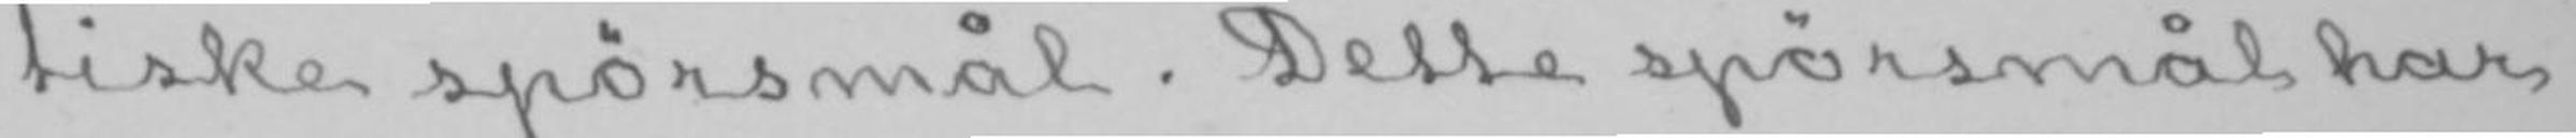tiske spørsmål. Dette spørsmål har
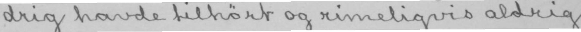drig havde tilhørt og rimeligvis aldrig
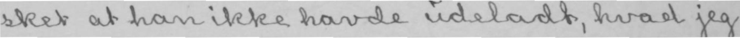sket at han ikke havde udeladt, hvad jeg
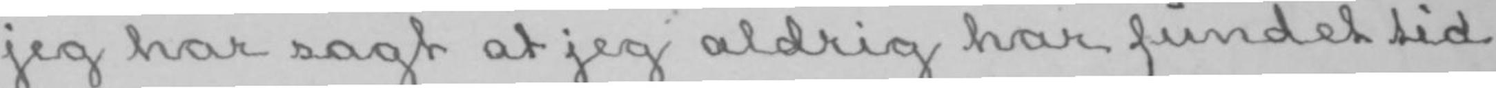jeg har sagt at jeg aldrig har fundet tid
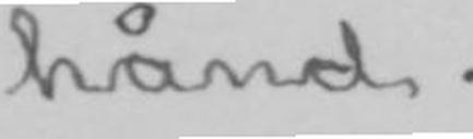hånd.
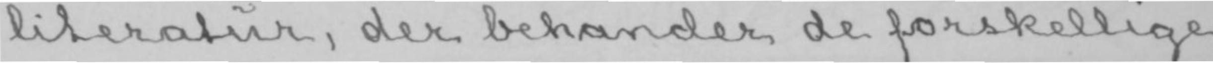literatur, der behander de forskellige
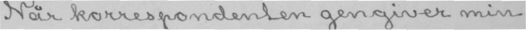Når korrespondenten gengiver min
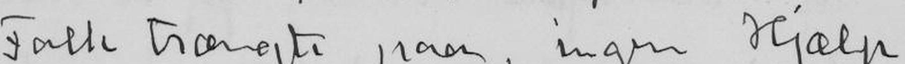Folk trængte paa, ingen Hjælp
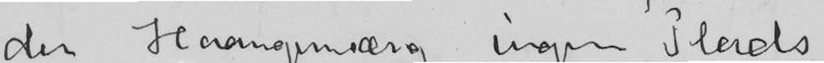der Haardgemæng ingen Plads,
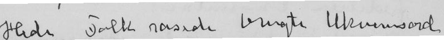Hede, Folk rasede brugte Ukvemsord
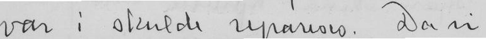var i skulde repareras. Da vi
